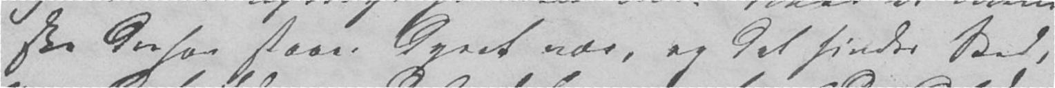ske derfor staaer Dyret nær, og det finder Sted,
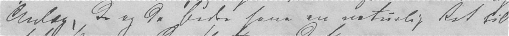Anlæg, de og de Bedre have en naturlig Ret til
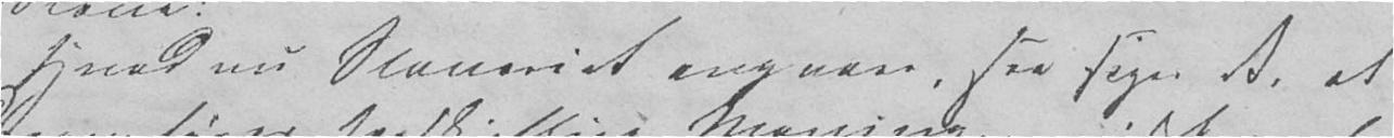Hvad nu Slaveriet angaar, saa siger A. at
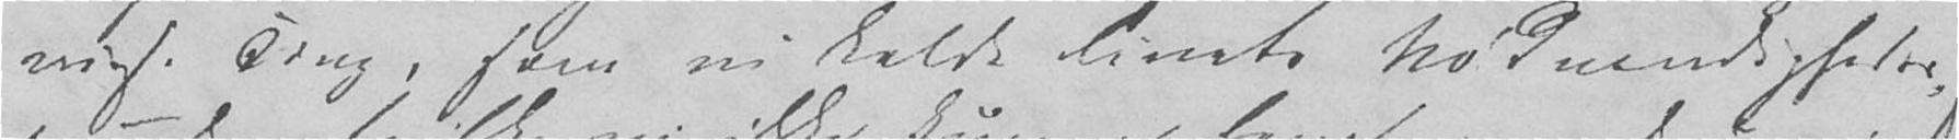visse Ting, som vi kalde Livets Nødvendigheder,
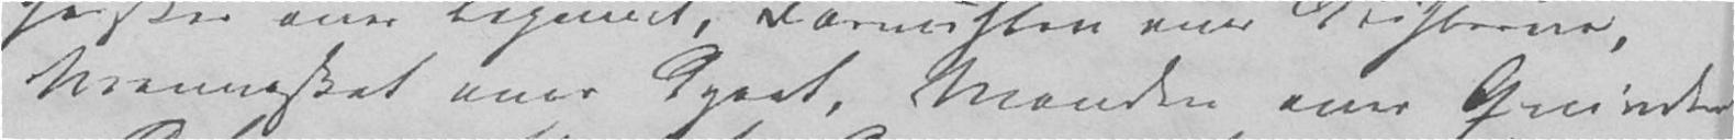Mennesket over Dyret, Manden over Qvinden
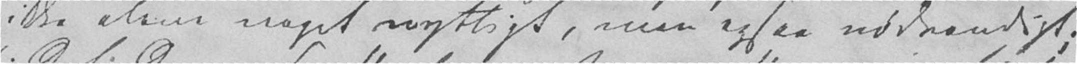ikke allene noget nyttigt, men ogsaa nødvendigt;
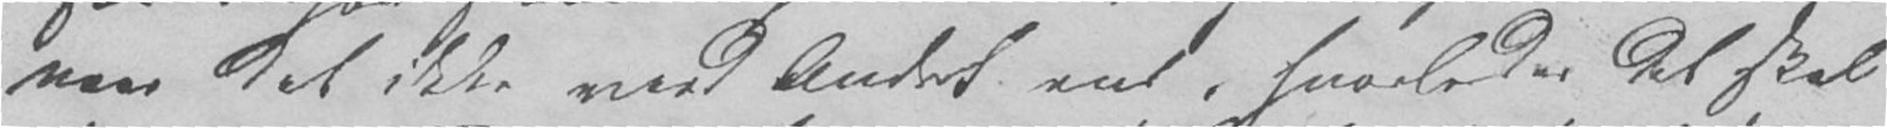naar det ikke veed Andet end, hvorledes det skal
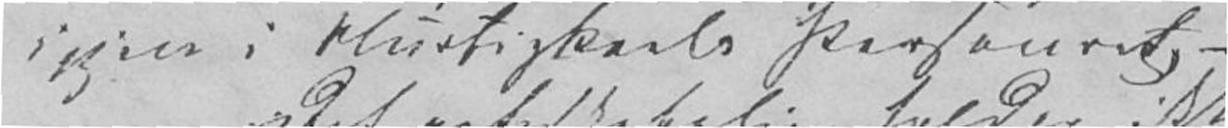igjen i Hurtigkarls Personret,-
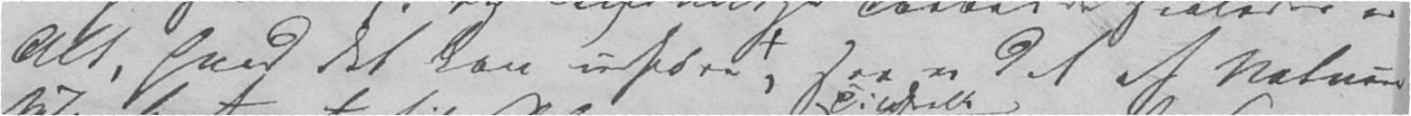Alt, hvad det kan udføre, saa er det af Naturen
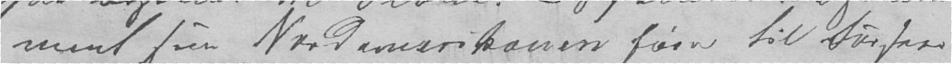ment som Nordamerikanere fører til Forsvar
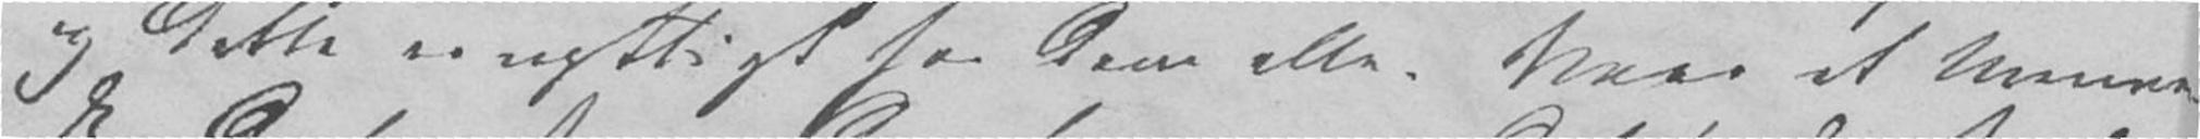og dette er nyttigt for dem alle. Naar et Menne-
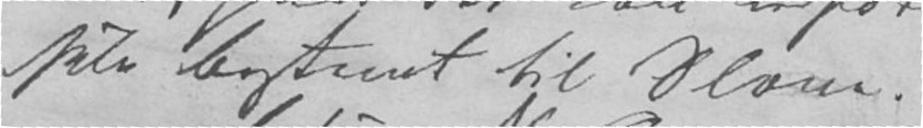selv bestemt til Slave.
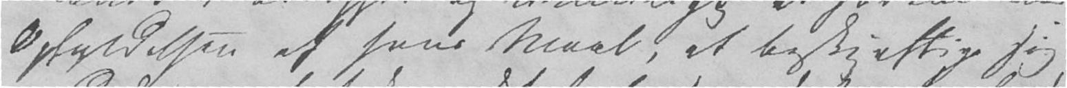Opfyldelsen af hans Maal, at beskjæftige sig
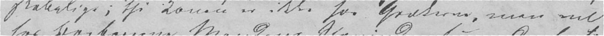skabelige; thi Konen er ikke hos Grækerne, men vel
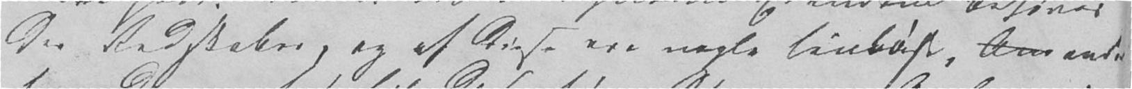der Redskaber, og af disse ere nogle livløse, An andre
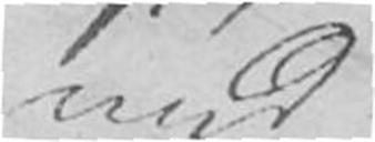med
